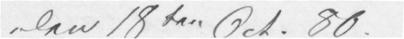Den 18te Oct. 80.
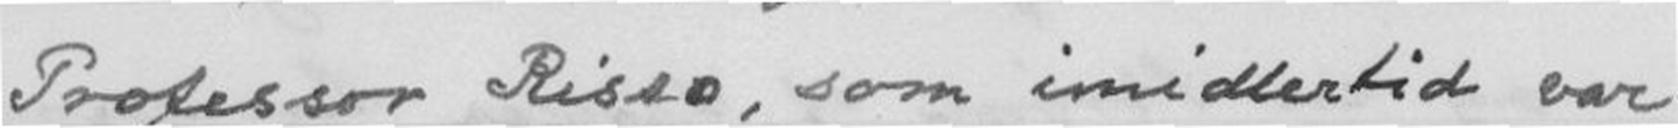Professor Risso, som imidlertid var
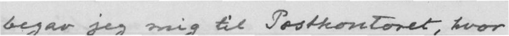begav jeg mig til Postkontoret, hvor
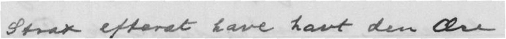Strax efterat have havt den Ære
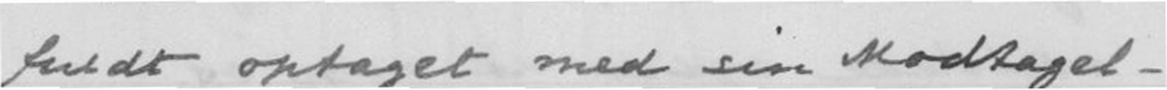fuldt optaget med sin Modtagel-
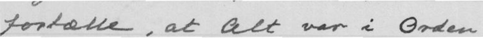fortælle, at Alt var i Orden
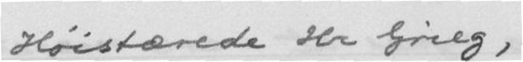Høistærede Hr Grieg,
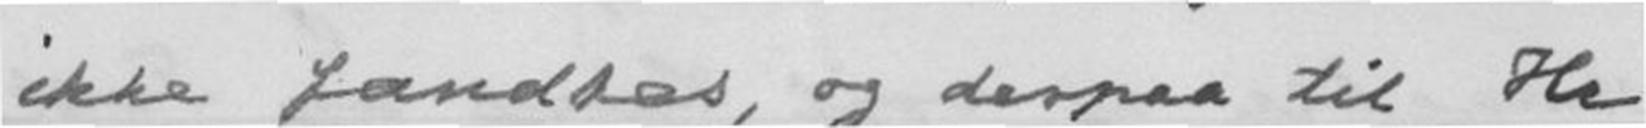ikke fandtes, og derpaa til Hr
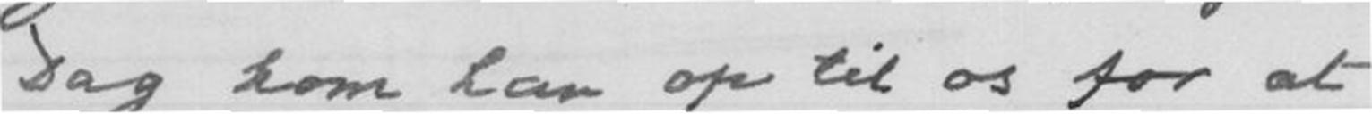Dag kom han op til os for at
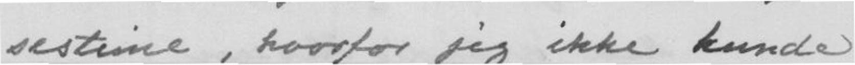sestime, hvorfor jeg ikke kunde
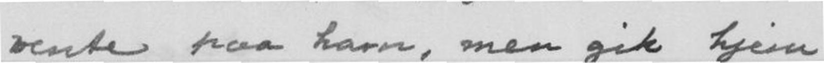vente paa ham, men gik hjem
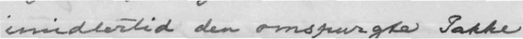imidlertid den omspurgte Pakke
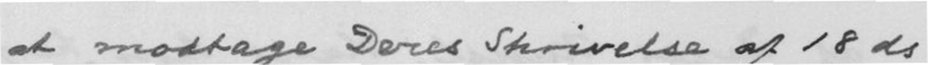at modtage Deres Skrivelse af 18 ds
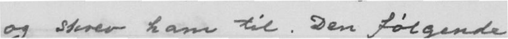og skrev ham til. Den følgende
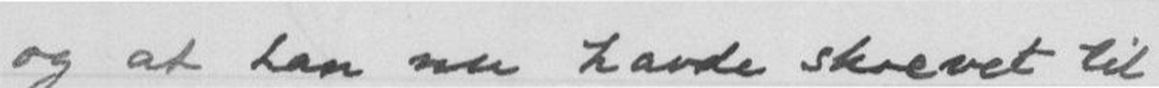og at han nu havde skrevet til
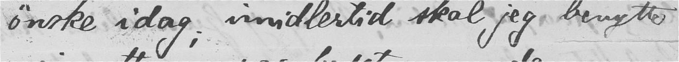ønske idag; imidlertid skal jeg benytte
